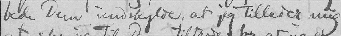bede Dem undskylde, at jeg tillader mig
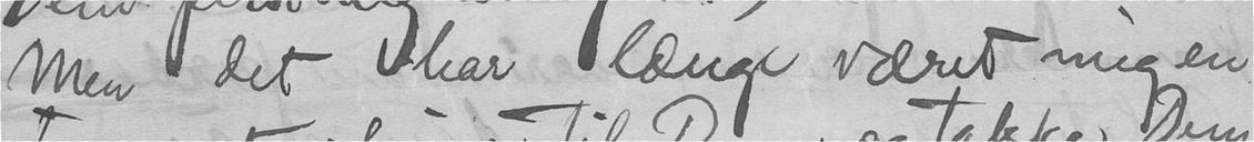Men det har længe været mig en
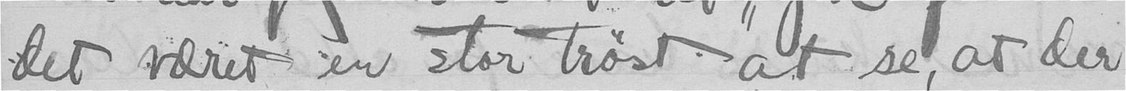det været en stor trøst - at se, at der
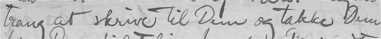trang at skrive til Dem og takke Dem
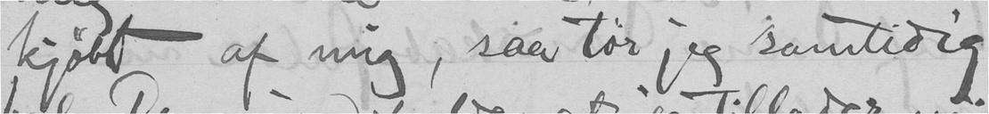kjøbt af mig, saa tør jeg samtidig
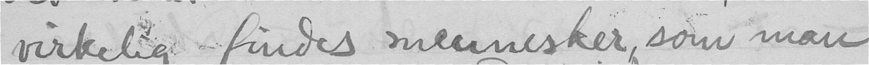virkelig - findes mennesker som man
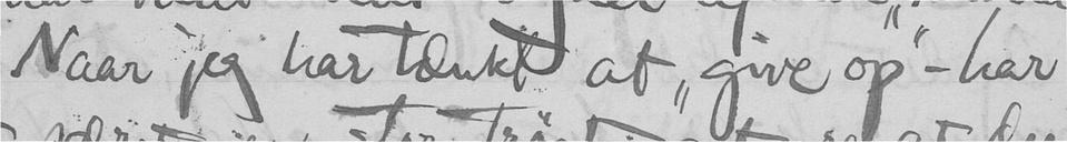Naar jeg har tænkt at "give op" - har
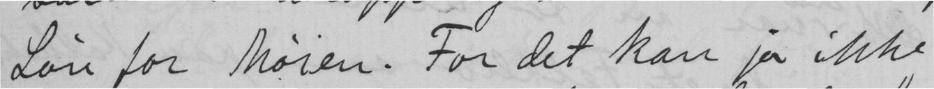Løn for Møien. For det kan jo ikke
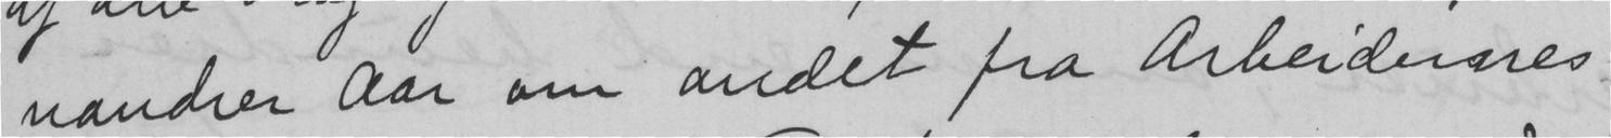vandrer aar om andet fra Arbeidernes
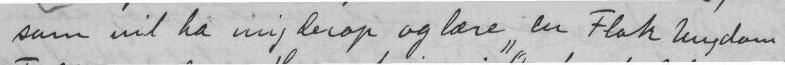som vil ha mig derop og lære en Flok Ungdom
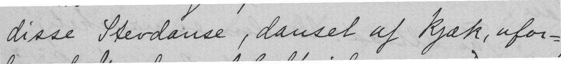disse Stevdanse, danset af Kjæk, ufor-
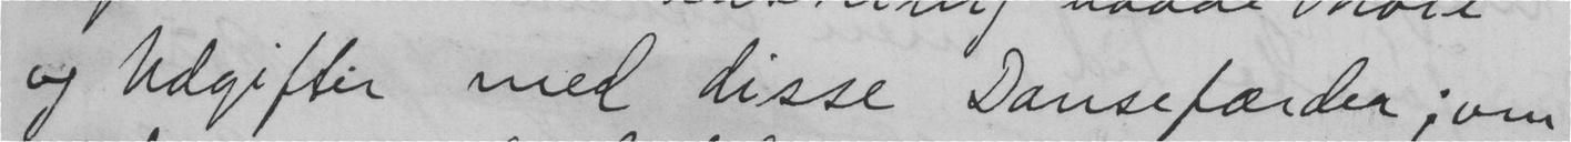og Udgifter med disse Dansefærder; om
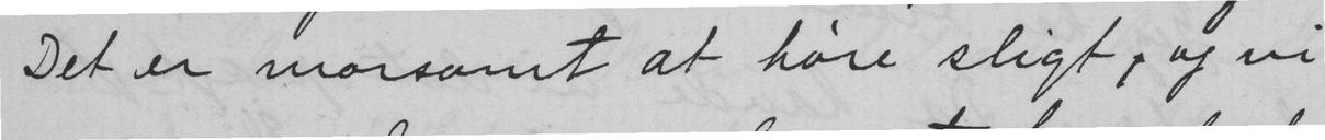Det er morsomt at høre sligt, og vi
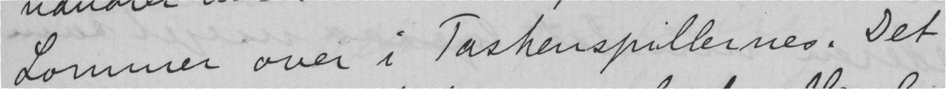Lommer over i Taskenspillernes. Det
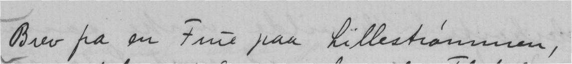Brev fra en Frue paa Lillestrømmen,
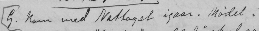G. kom med Nattoget igaar. Mødet i
